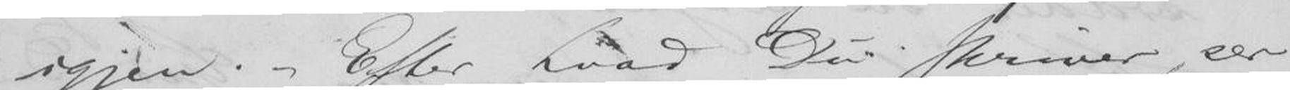igjen. - Efter hvad Du skriver, ser
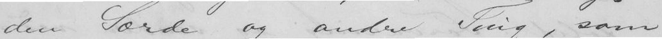den Særde og andre Ting, som
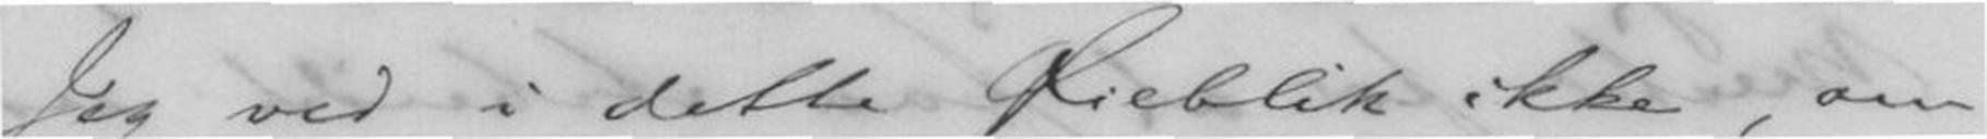Jeg ved i dette Øieblik ikke, om
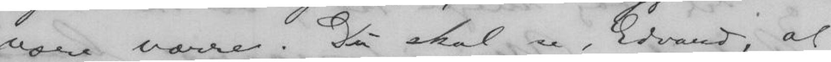være værre. Du skal se, Edvard, at
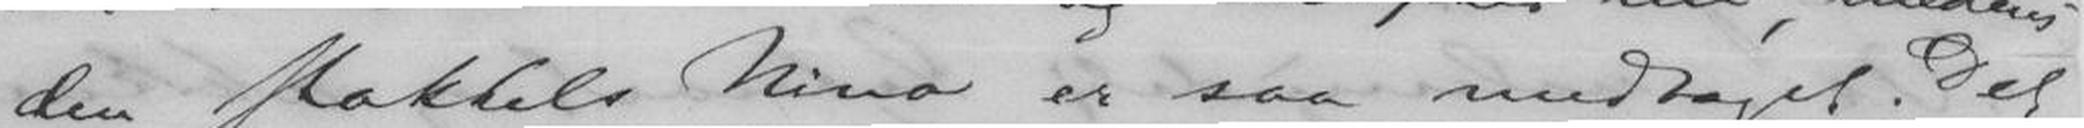den stakkels Nina er saa medtaget. Det
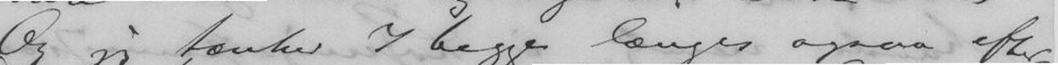Og jeg tænker I begge længes ogsaa efter
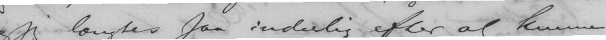jeg længtes saa inderlig efter at kunne
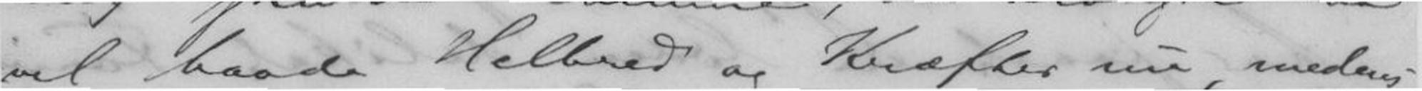vel baade Helbred og Kræfter nu, medens
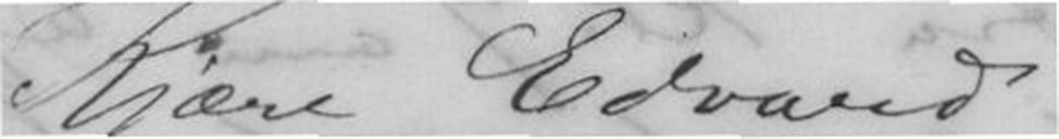Kjære Edvard!
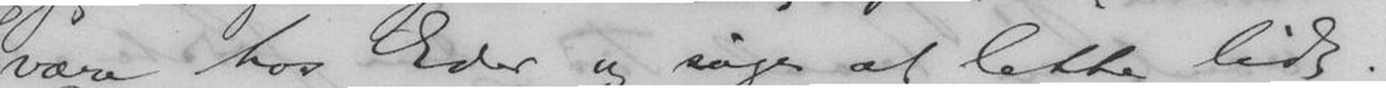være hos Eder og søge at lette lidt.
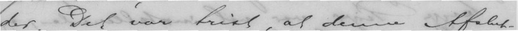der. Det var trist, at denne Afslut-
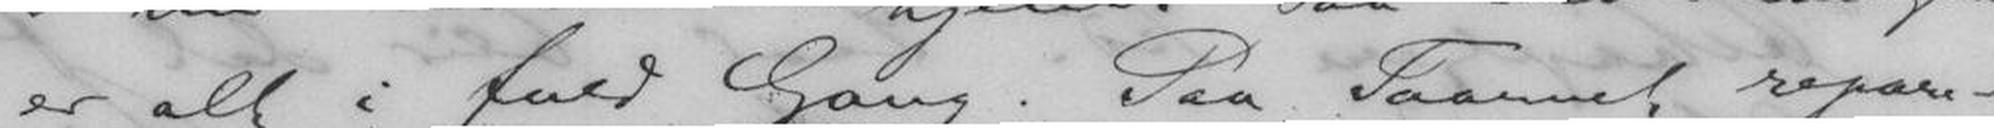er alt i fuld Gang. Paa Taarnet repare-
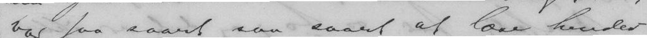var saa saart saa saart at læse hendes
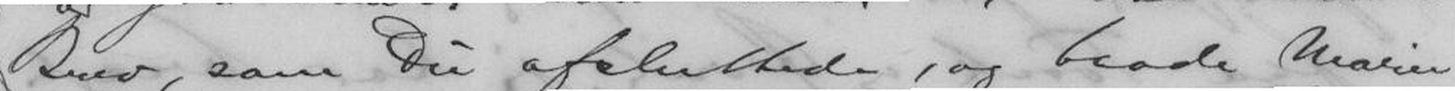Brev, som Du afsluttede, og baade Marie
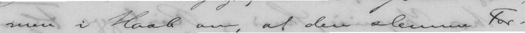men i Haab om, at den slemme For-
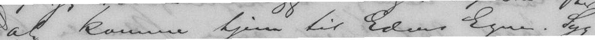at komme hjem til Eders Egne. Syg
